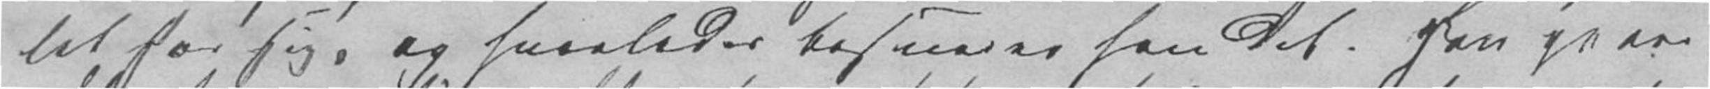let for sig, og saaledes besvarer han det. Han gaaer
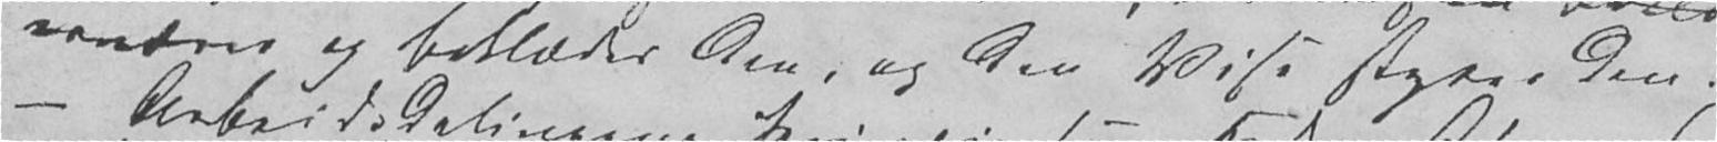vendrer og beklæder den, og den Vise styrer den.
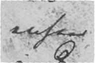enhver
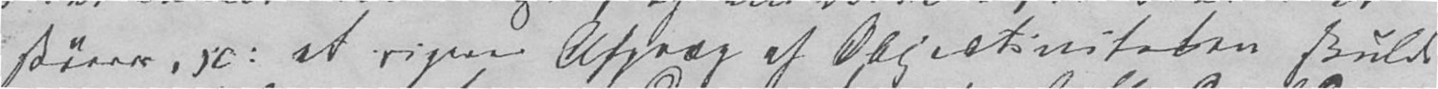skreve, : et rigere Afpræg af Obejctiviteten skulde
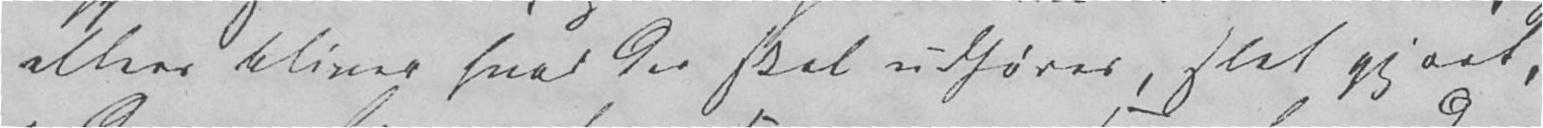ellers bliver hvad der skal udføres, slet gjort,
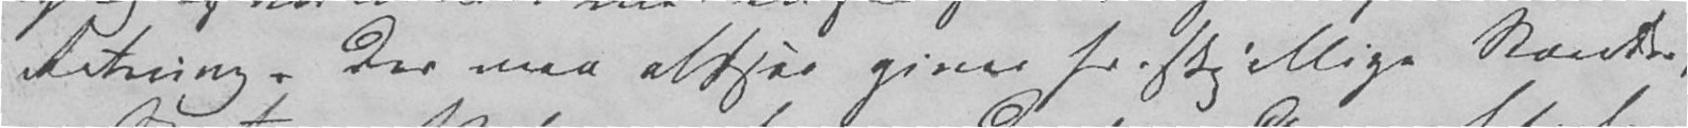Retning. Der maa altsaa gives forskjellige Stænder,
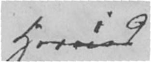Herimod
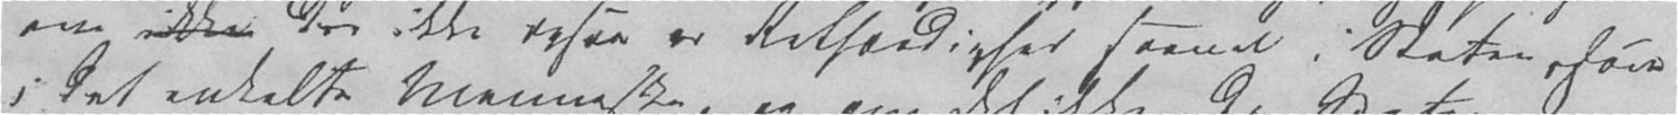om ikke der ikke ogsaa er Retfærdighed saavel i Staten, som
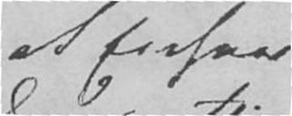at Enhver
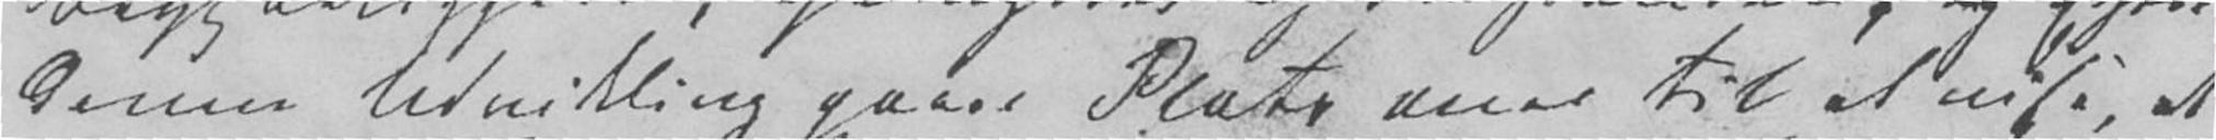denne Udvikling gaaer Platon over til at vise, at
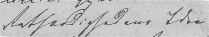Retfærdighedens Idee
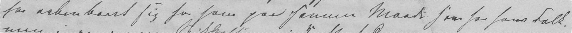har aabenbaret sig for ham paa samme Maade som hos hans Folk.
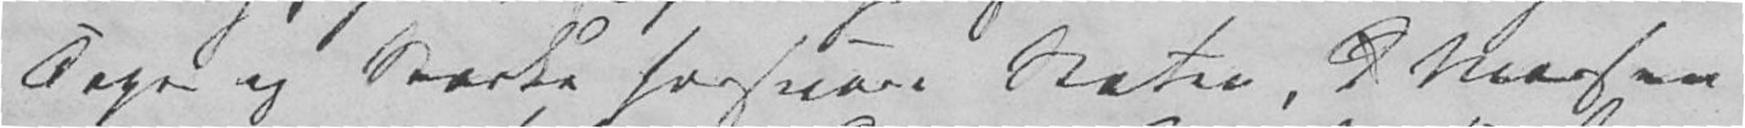Sage og Stærke forsvare Staten, d Massen
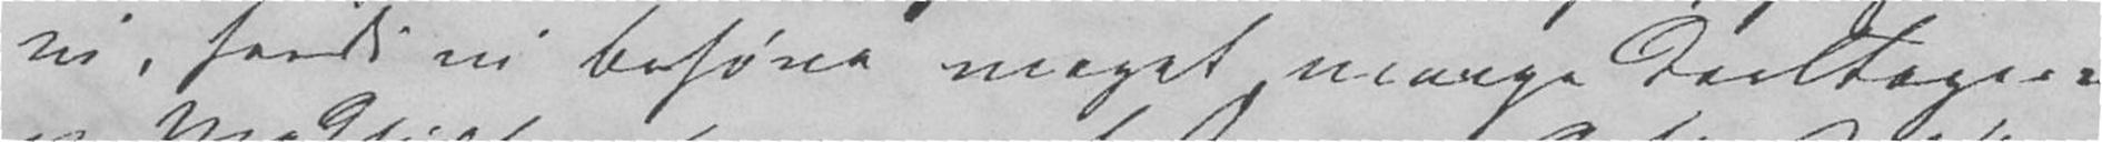vi, fordi vi behøve meget, mange Deeltagere
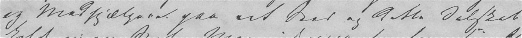og Medhjælpere paa eet Sted og dette Selskab
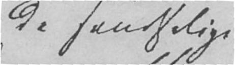de sandselige
